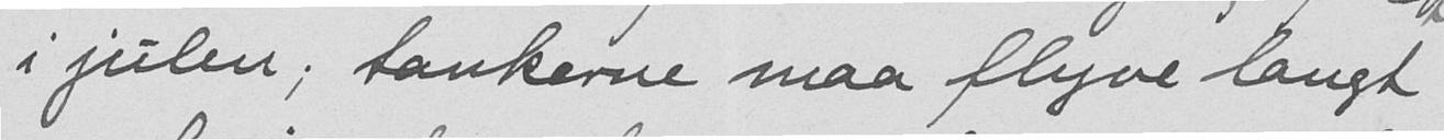i julen; tankerne maa flyve langt
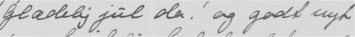Glædelig jul da! og godt nyt
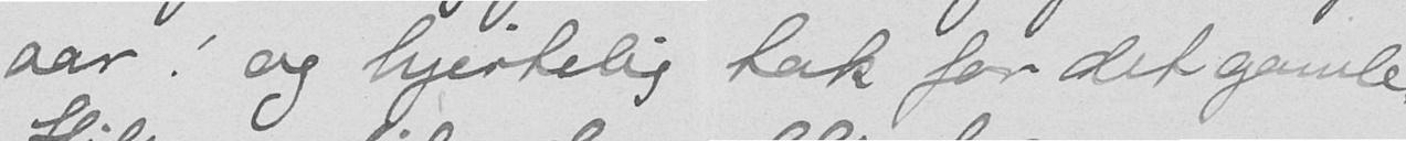aar! og hjertelig tak for det gamle
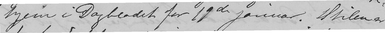hjem i Dagbladet for 19de januar. Stilen er
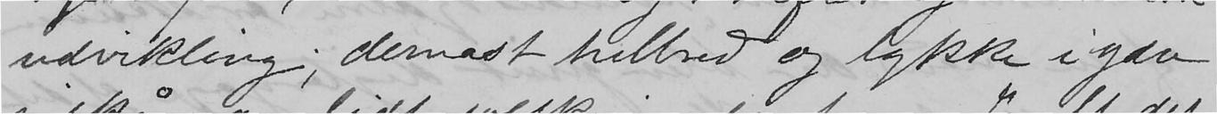udvikling, dernæst helbred og lykke ydre
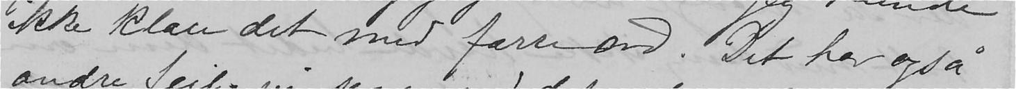ikke klare det med farre ord. Det har også
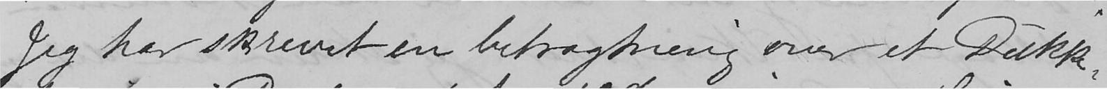Jeg har skrevet en betragtning over et Dukk
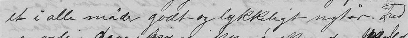et i alle måder godt og lykkeligt nytår. Fred
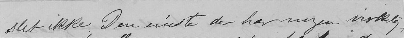slet ikke. Den eneste der har nogen virkelig-
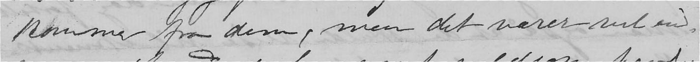kommer fra dem, men det varer vel end-
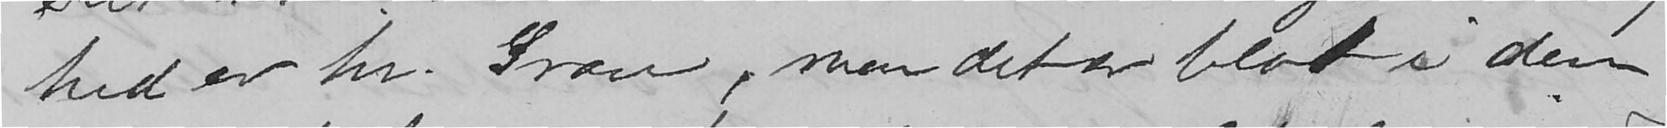hed er hr. Gran, men det er blot i den
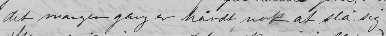det mangen gang er hårdt nok at slå sig
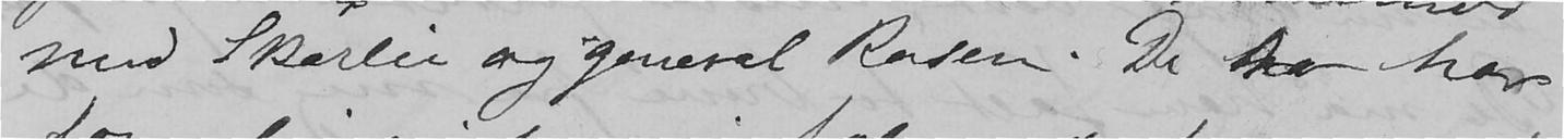med Skarlie og general Rosen. De to har
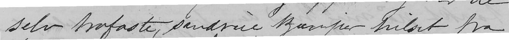selv trofaste, sandrue kjæmper hilset fra
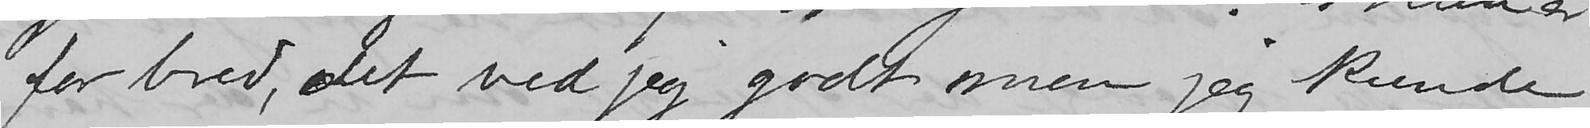for bred, det ved jeg godt men jeg kunde
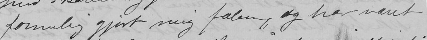formlig gjort mig falen, og har været
